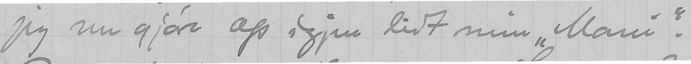jeg nu gjøre op igjen lidt min "Mani".
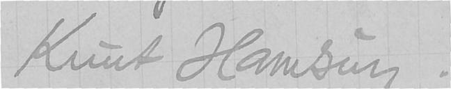Knut Hamsun.
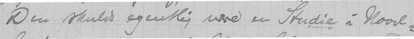Den skulde egentlig være en Studie i Novel-
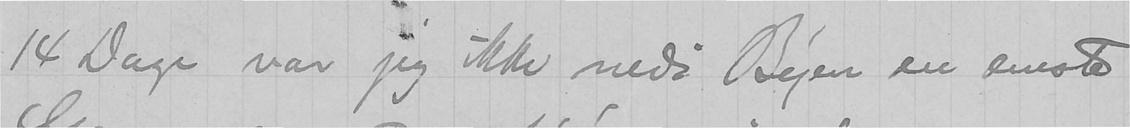14 Dage var jeg ikke nedi Byen en eneste
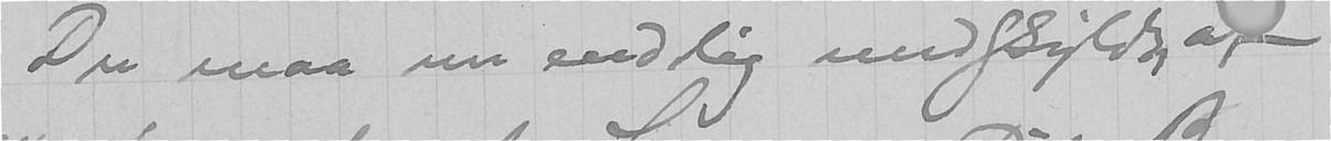Du maa nu endlig undskylde at
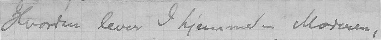Hvordan lever I hjemme - Moderen,
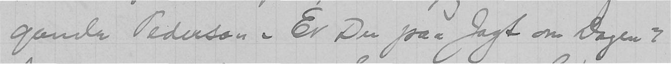gamle Pederson. Er Du paa Jagt om Dagen?
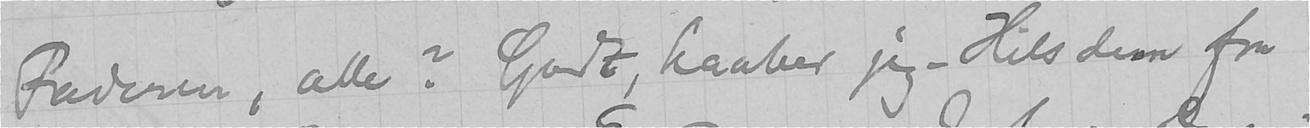Faderen, alle? Godt, haaber jeg - Hils dem fra
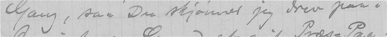Gang, saa Du skjønner jeg drev paa.
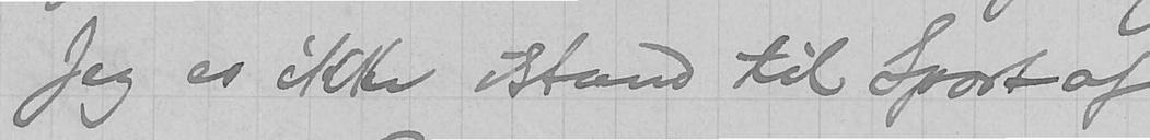Jeg er ikke istand til Sport af
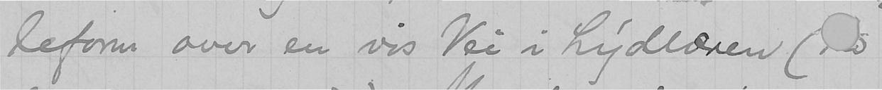leform over en vis Vei i Lydlæren (
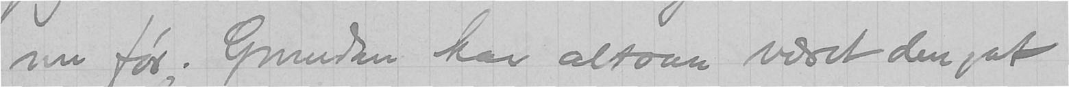nu før. Grunden har altsaa været den, at
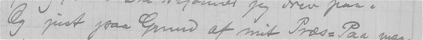Og just paa Grund af mit Præs-Paa maa
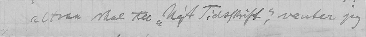altsaa skal til "Nyt Tidsskrift", venter jeg
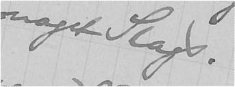noget Slags.
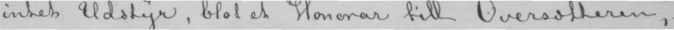intet Udstyr, blot et Honorar till Oversætteren,-
